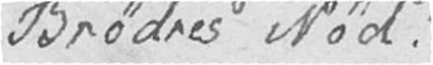Brødres Nød!
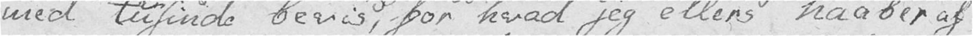med tusinde bevis, for hvad jeg ellers haaber af
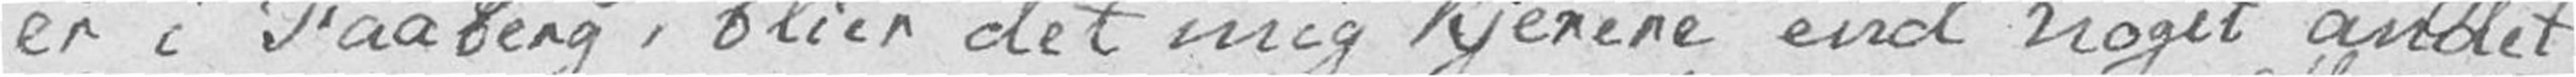er i Faaberg, blier det mig Kjerere end noget andet
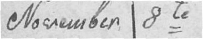November 8te
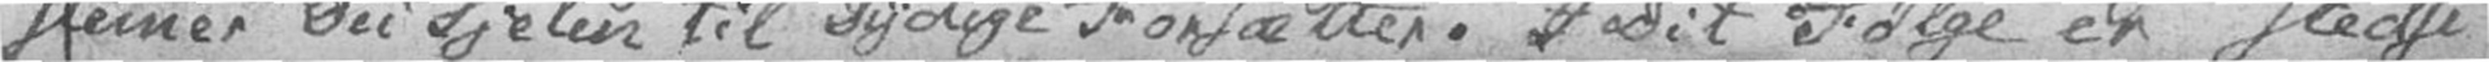stemmer Du Sjelen til Dydige Forsætter. I dit Følge er stedse
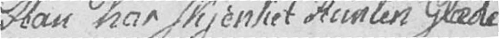Han har skjenket Himlen Glæde
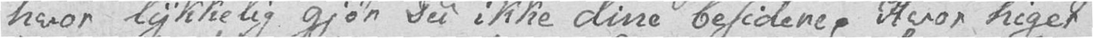hvor lykkelig gjør Du ikke dine besidere, Hvor higer
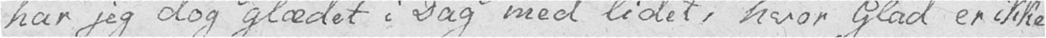har jeg dog glædet i Dag med lidet, hvor Glad er ikke
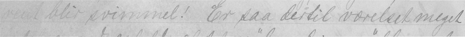rent blir svimmel! Er saa dertil værelset meget
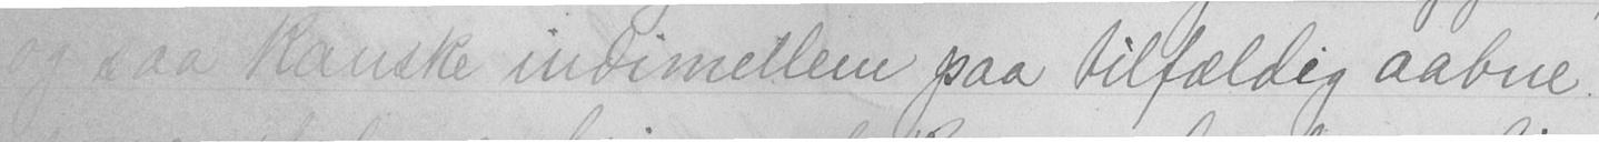og saa kanske indimellem paa tilfældig aabne
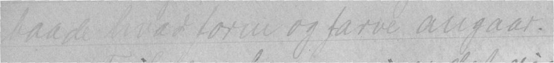baade hvad form og farve angaar.
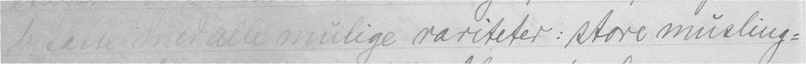besatte med alle mulige rariteter: store musling-
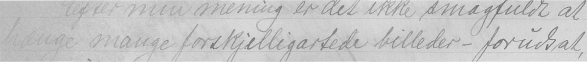hænge mange forskjelligartede billeder - forudsat,
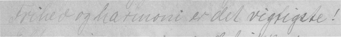Frihed og harmoni er det vigtigste!
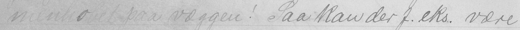menholet paa væggen! Saa kan der f. eks. være
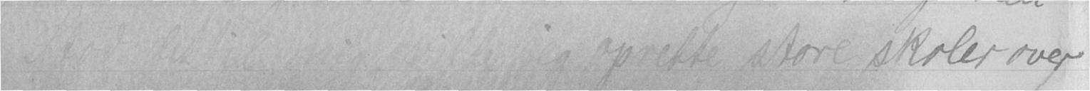Stod det op til mig skulle jeg oprette store skole over
